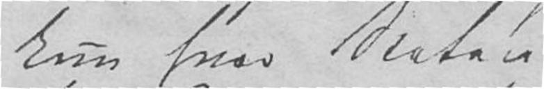kun hvor Staten
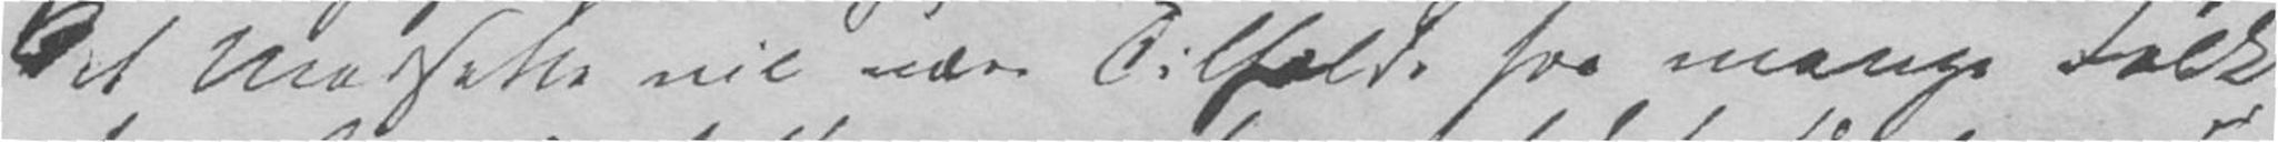det Modsatte vil være Tilfælde hos mange Folk
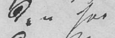den har
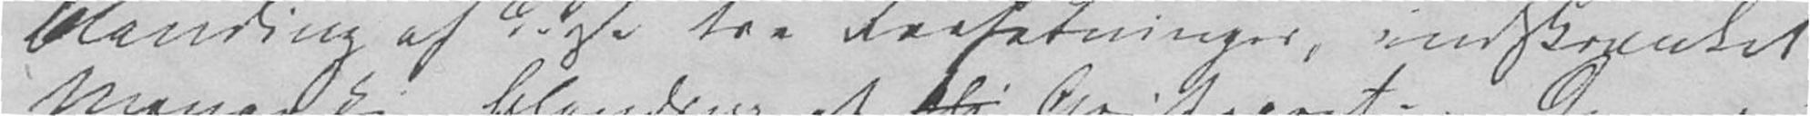Blanding af disse tre Forfatninger, indskrænket
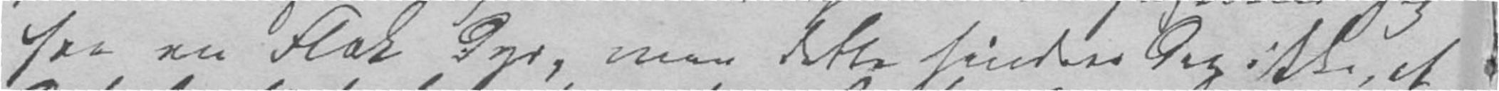fra en Flok Dyr, men dette hindrer dog ikke, at
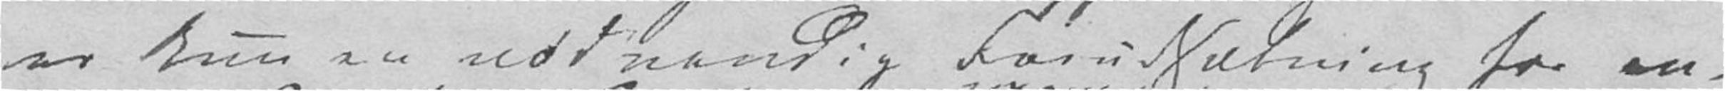er kun en nødvendig Forudsætning for en-
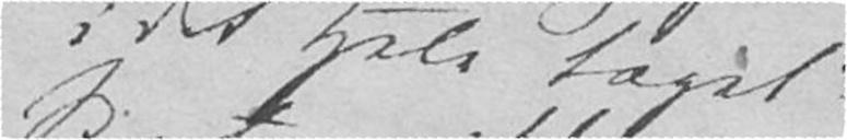i det hele taget
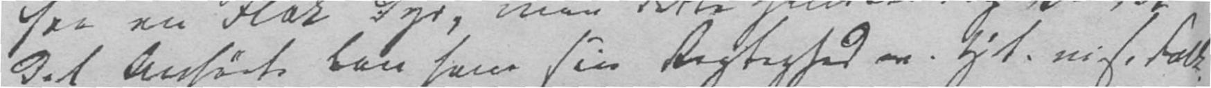det Anførte kan have sin Rigtighed. Hist. visse Folk-
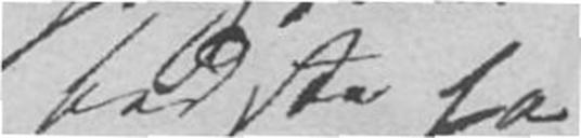Bedste for
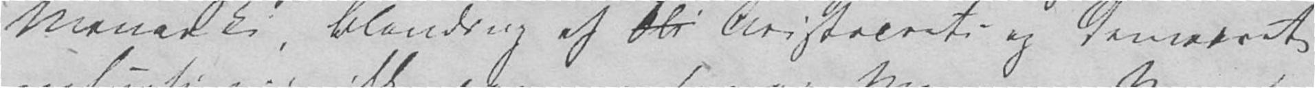Monarki, Blanding af Oli Aristocrati og Deomcrati
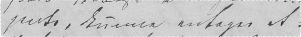gents, kunne antages at
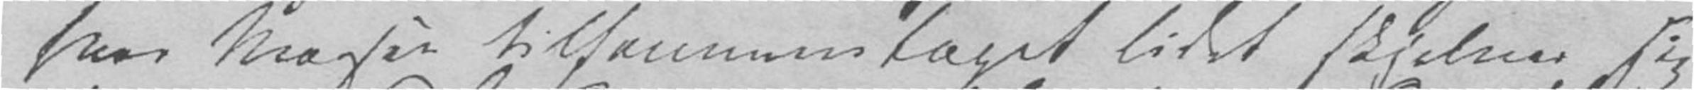hvor Massen tilsammentaget lidet skjelner sig
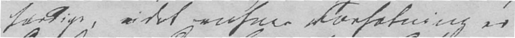færdige, idet enhver Forfatning er
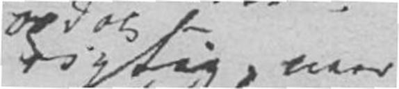rigtig, naar
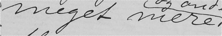meget mere
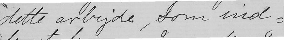dette arbejde, som ind-
